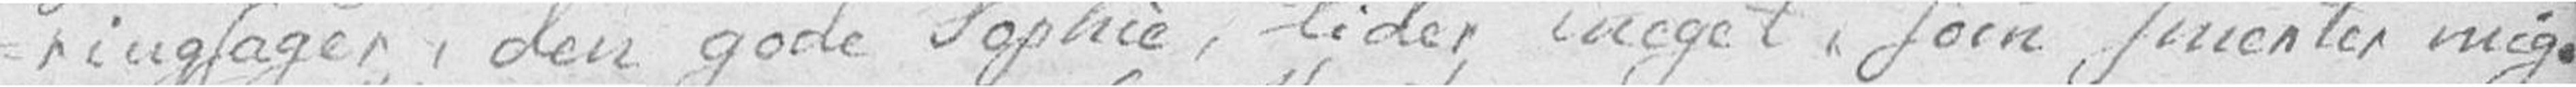-ringsager, den gode Sophie, lider meget, som smerter mig.
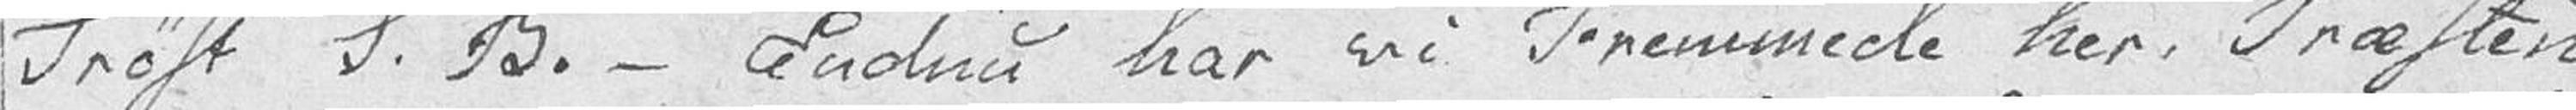Trøst S. B. – Endnu har vi Fremmede her. Præsten
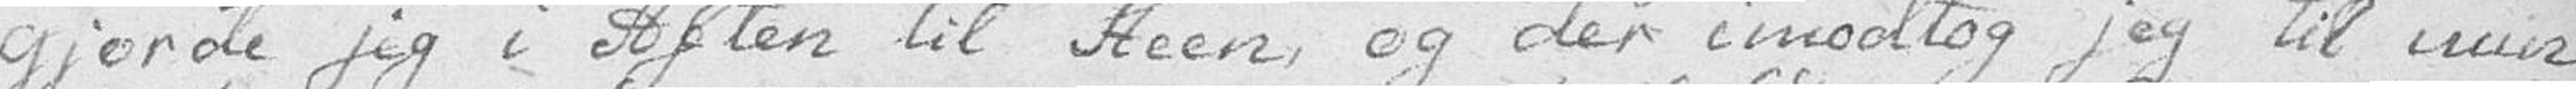gjorde jeg i Aften til Steen, og der imodtog jeg til min
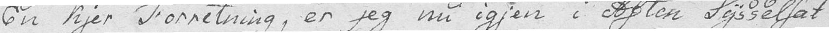En kjer Forretning, er jeg nu igjen i Aften Sysselsat
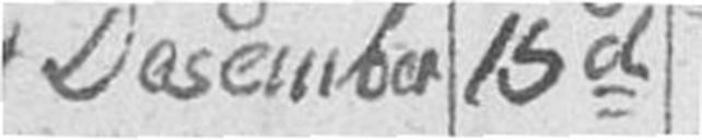Desember 15d
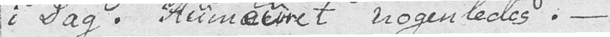i Dag. Humeuret nogenledes. –
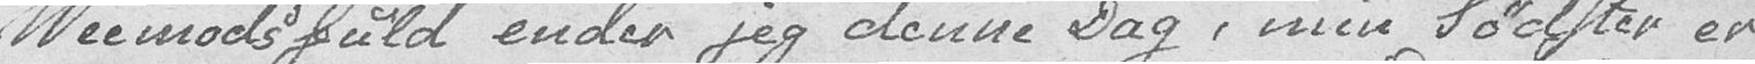Veemodsfuld ender jeg denne Dag, min Sødster er
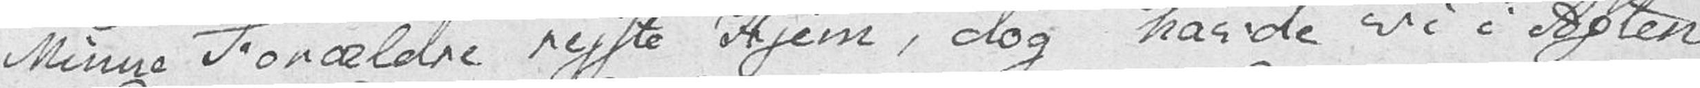Minne Forældre reiste Hjem, dog havde vi i Aften
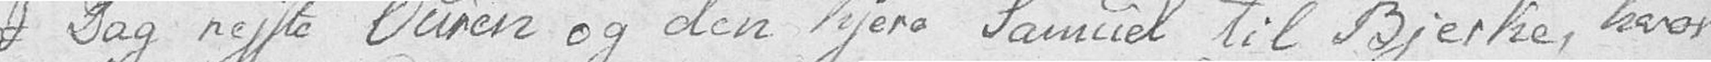I Dag reiste Ouren og den kjere Samuel til Bjerke, hvor
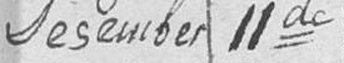Desember 11de
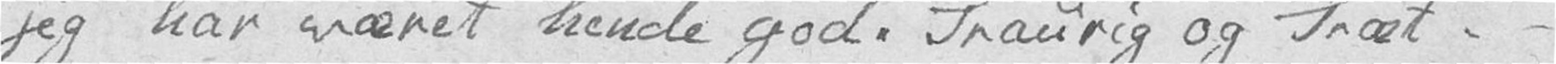jeg har været hende god. Traurig og Træt. –
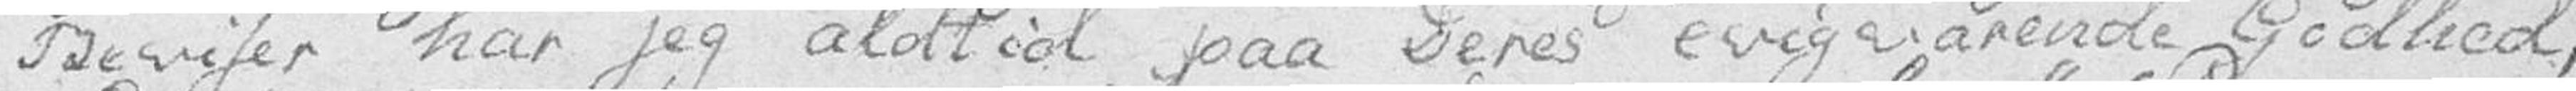Beviser har jeg aldtid paa Deres evigvarende Godhed,
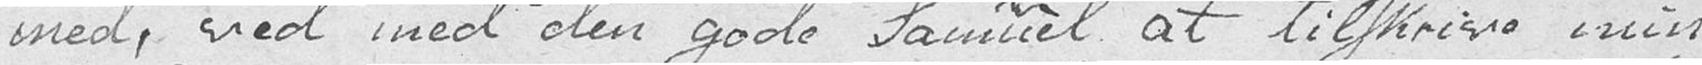med, ved med den gode Samuel at tilskrive min
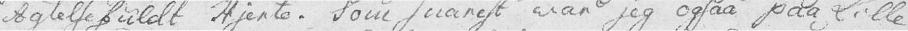Agtelsefuldt Hjerte. Som snarest var jeg ogsaa paa Lille
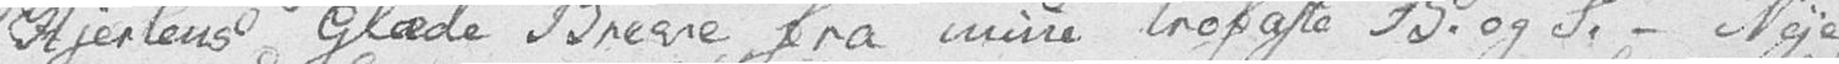Hjertens Glæde Breve fra mine trofaste B. og S. – Nøje
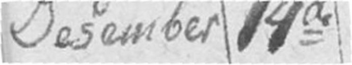Desember 14de
